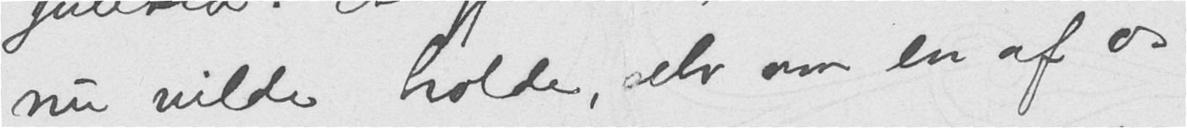nu vilde holde, selv om en av os
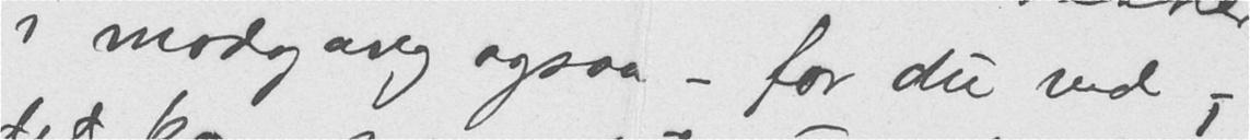i modgang ogsaa - for du ved, -
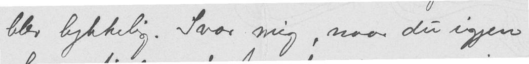blev lykkelig. Svar mig, naar du igjen
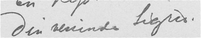Din veninde Sigrid.
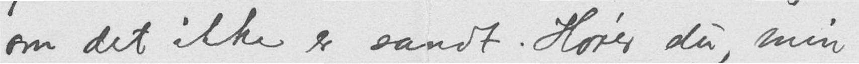om det ikke er sandt. Hør du, min
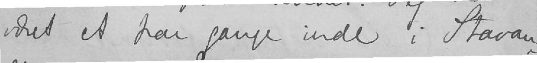været et par gange inde i Stavan-
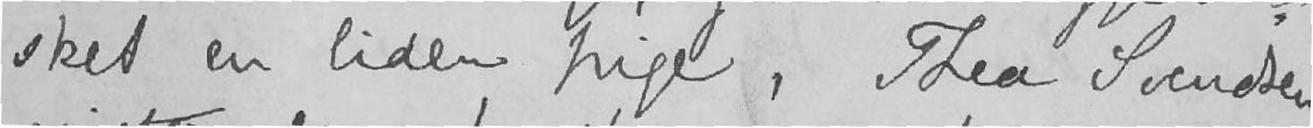sket en liden pige, Thea Svendsen
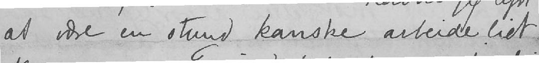at være en stund kanske arbeide lidt
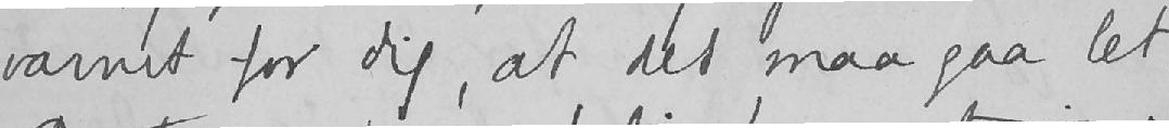varmt for dig, at det maa gaa let.
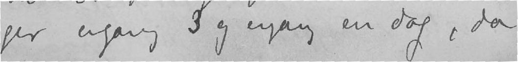ger engang 3 og engang en dag, da
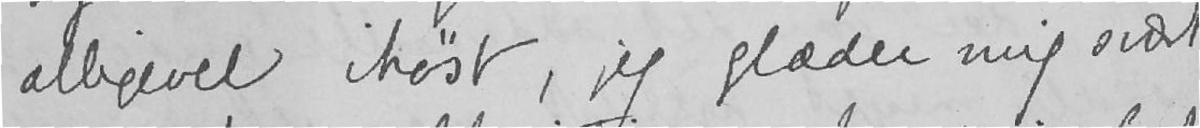alligevel ihøst, jeg glæder mig svært
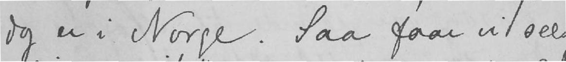og er i Norge. Saa faar vi sees
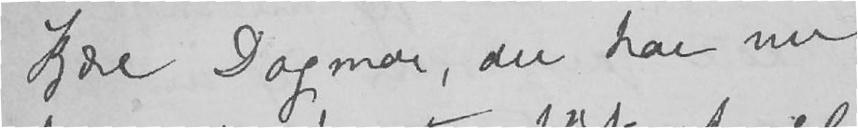Kjære Dagmar, du har nu
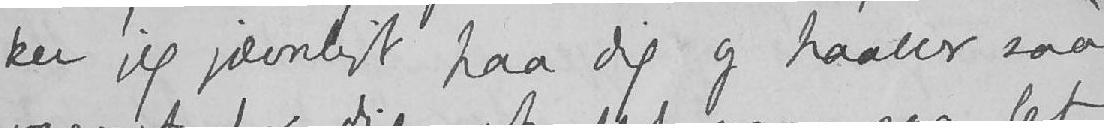ker jeg jævnligt paa dig og haaber saa
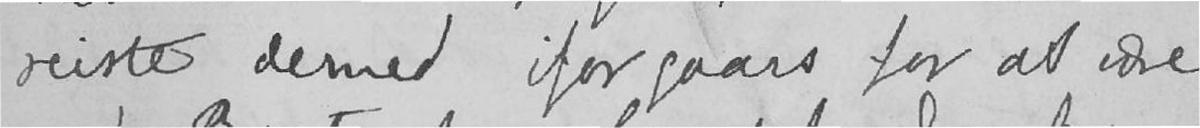reiste derned iforgaars for at være
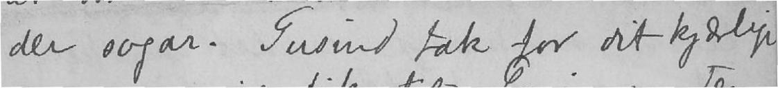der sogar. Tusind tak for dit kjærlige
